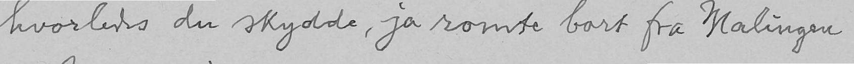hvorledes du skydde, ja romte bort fra Malingen
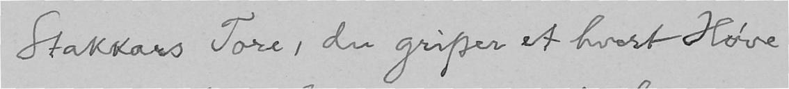Stakkars Tore, du griper et hvert Høve
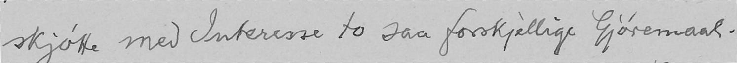skjøtte med Interesse to saa forskjellige Gjøremaal.
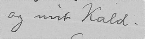og mit Kald.
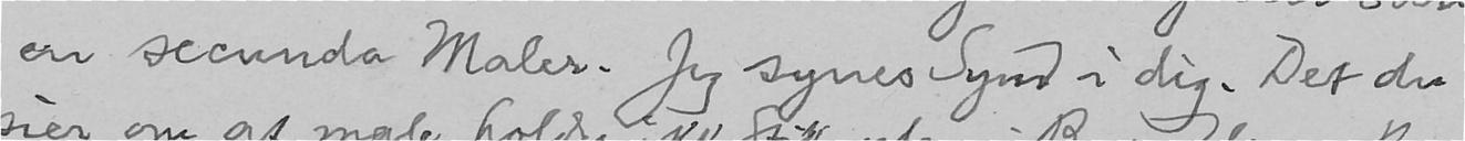en secunda Maler. Jeg synes Synd i dig. Det du
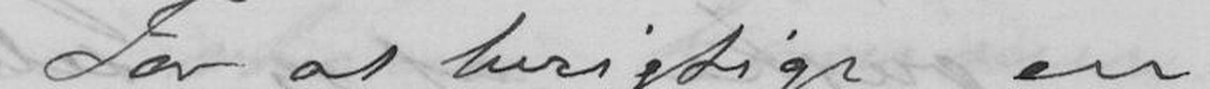For at berigtige en
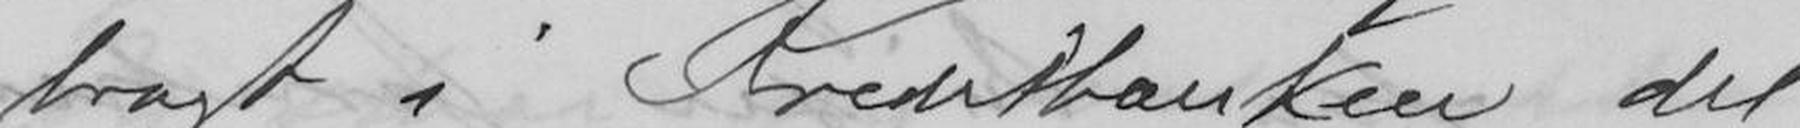bragt i Kreditbanken det
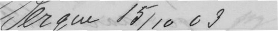Bergen 15/10 03
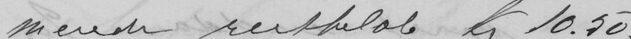mende restbeløb kr 10.50.
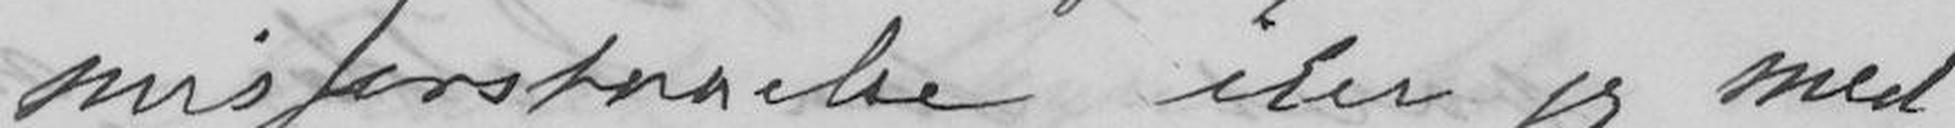misforstaalse iler jeg med
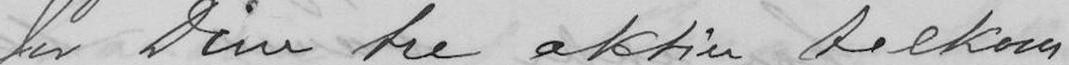for Dine tre aktier tilkom-
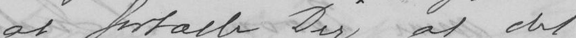at fortælle Dig, at det
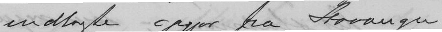indlagte opgjør fra Stavanger
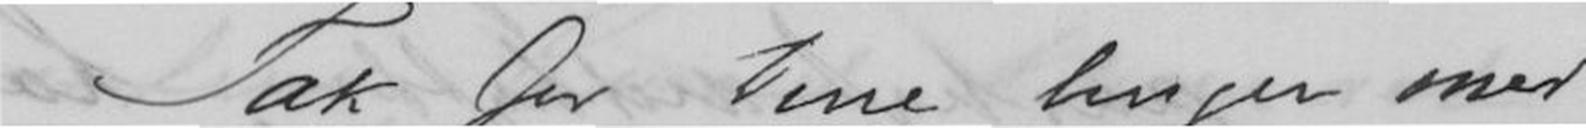Tak for Dine linjer med
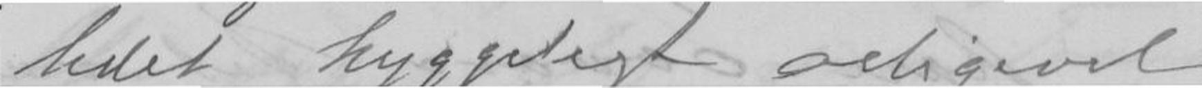ledet hyggeligt alligevel.
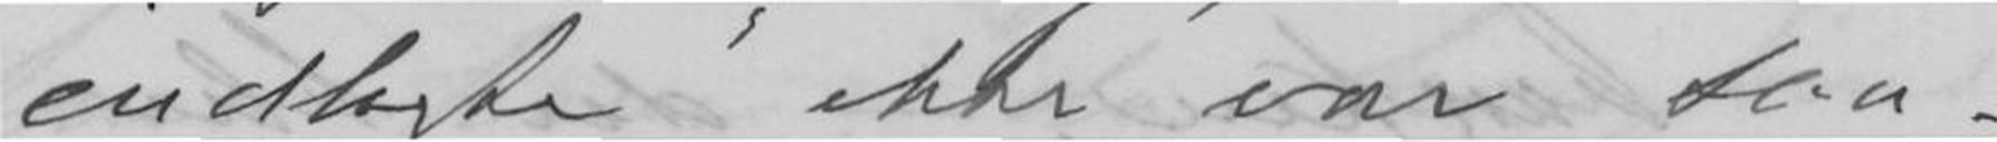indlagte ikke var saa-
